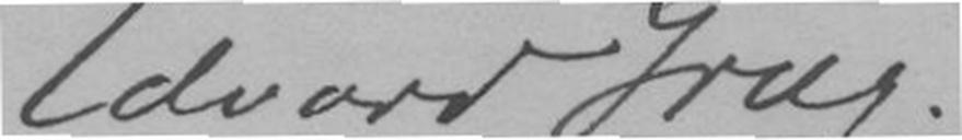Edvard Grieg!
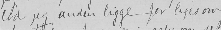lod jeg anden ligge for ligesom
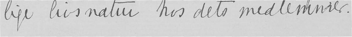lige livsnatur hos dets medlemmer.
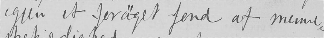igjen et forøget fond af menne-
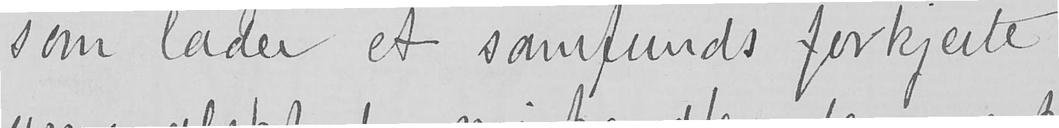som lader et samfunds forkjerte
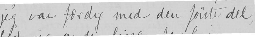jeg var færdig med den første del,
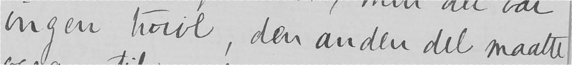ingen tvivl, den anden del maatte
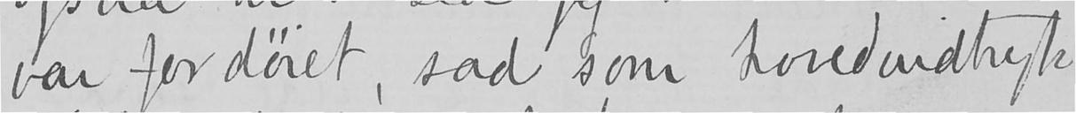var fordøiet, sad som hovedindtryk
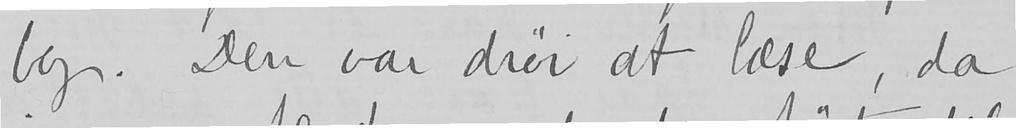bog. Den var drøi at læse, da
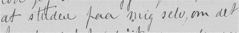at studere paa mig selv, om det
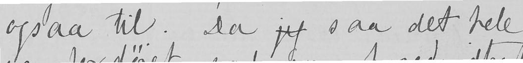ogsaa til. Da jeg saa det hele
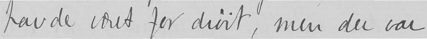havde været for drøit, men der var
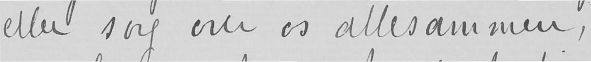eller sorg over os allesammen,
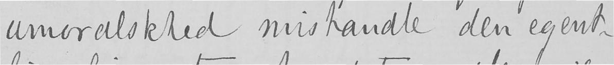umoralskhed mishandle den egent-
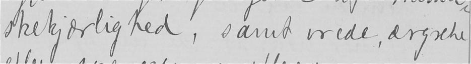skekjærlighed, samt vrede, ærgrelse
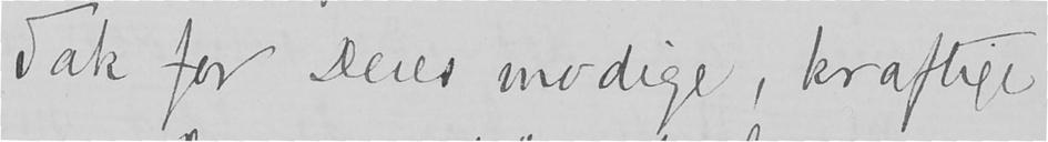Tak for Deres modige, kraftige
